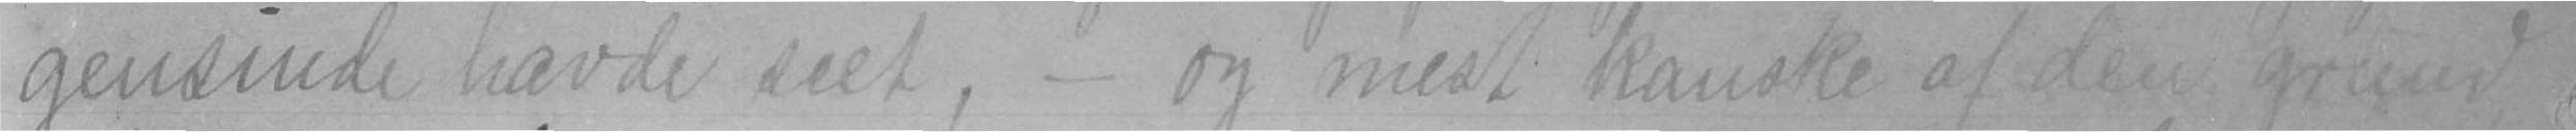gensinde havde seet, - og mest kanske af den grund
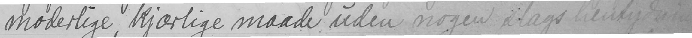moderlige, kjærlige maade uden nogen slags hentynin-
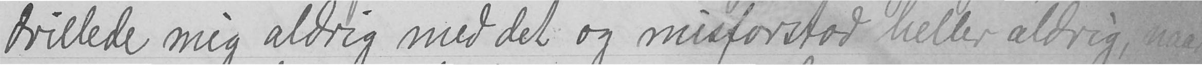drillede mig aldrig med det og misforstod heller aldrig, naar
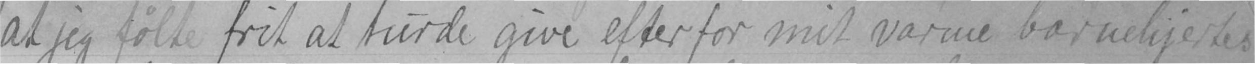at jeg følte frit at turde give efter for mit varme barnehjertes
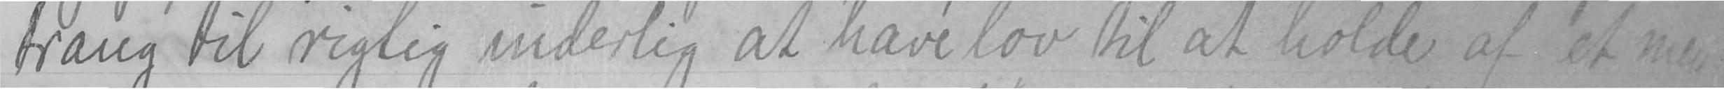trang til rigtig underlig at have lov til at holde af et men-
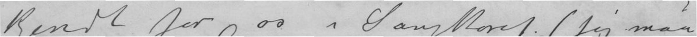kjendt for os i Sangkoret; (jeg maa
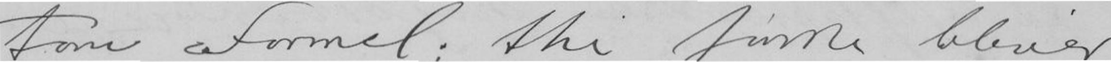Tone Formel; thi først bliver
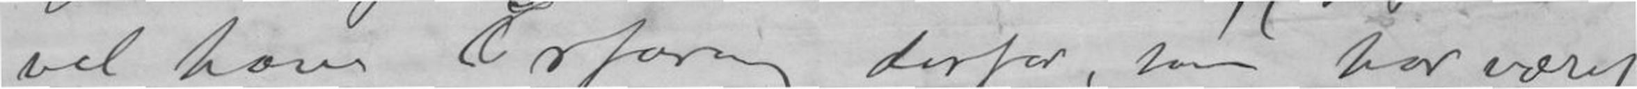vel have Erfaring derfor, som har været
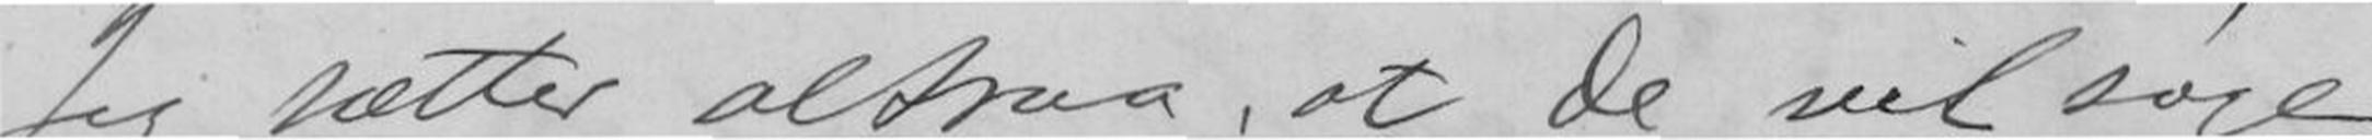Jeg sætter altsaa, at De vil søge
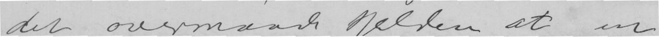det overmaade sjelden at en
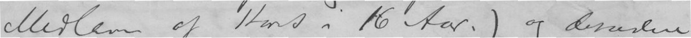Medlem af Hans i 16 Aar.) og desuden
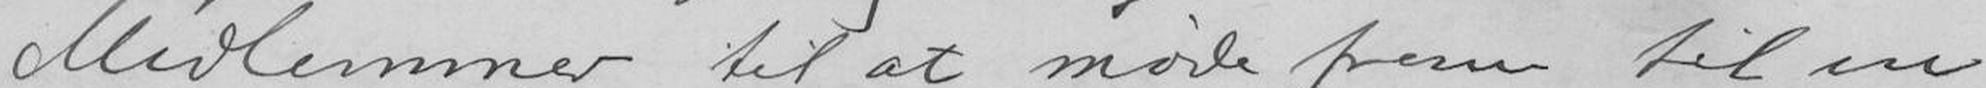Medlemmer til at møde frem til en
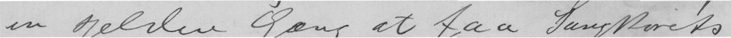en sjelden Gang at faa Sangkorets
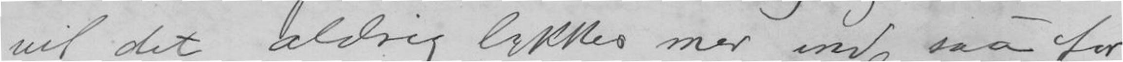vil det aldrig lykkes mer end saa for
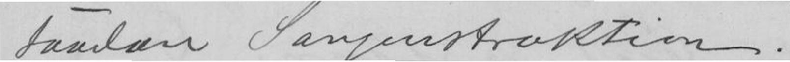saadan Sangenstraktion.
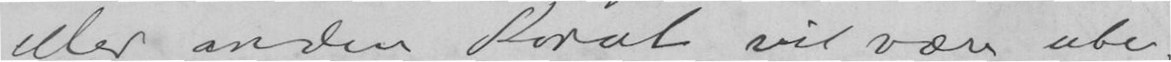eller anden Koral vil være ube-
